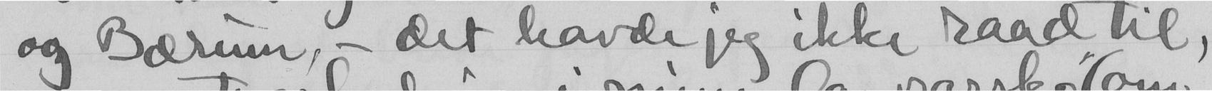og Bærum, - det havde jeg ikke raad til,
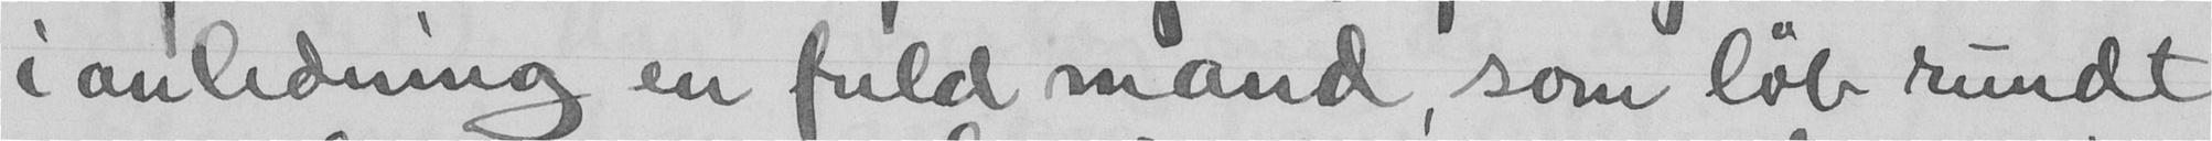i anledning en fulal mand som løb rundt
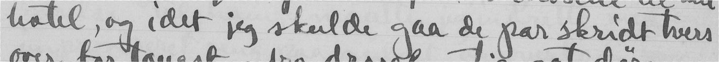hotel, og idet jeg skulde gaa de par skridt tvers
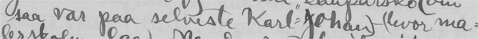laa var paa selveste Karl-Johan)- (hvor ma-
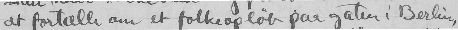at fortælle om et folkeopløb paa gaten i Berlin,
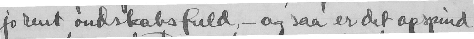jo rent ondskabsfuld, - og saa er det opspind
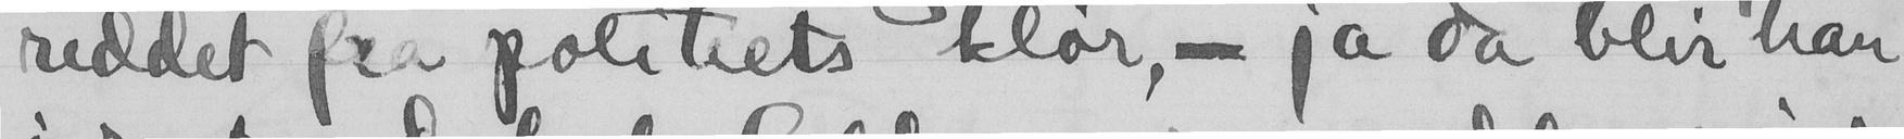reddet faa politiets klør, - ja da blir han
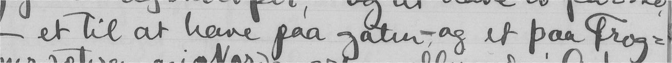- et til at have paa gaten, - og et paa Frog-
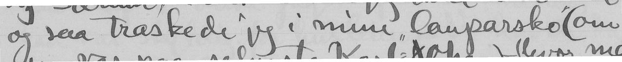os saa traskede jeg i mine "lauparsko" (om
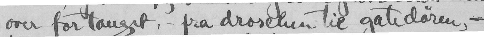over fortauget, - fra droschen til gatedøren, -
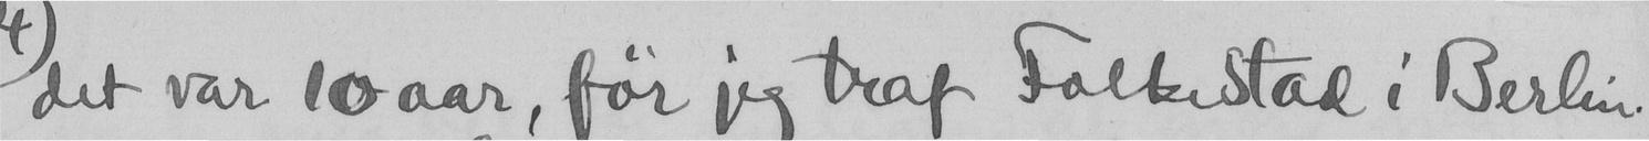det var 10 aar, før jeg traf Folkestad i Berlin.
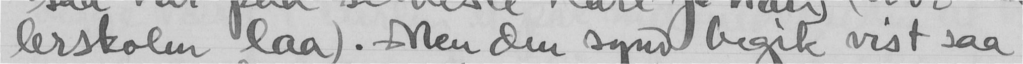lerskolen laa). Men den synd begik vist saa
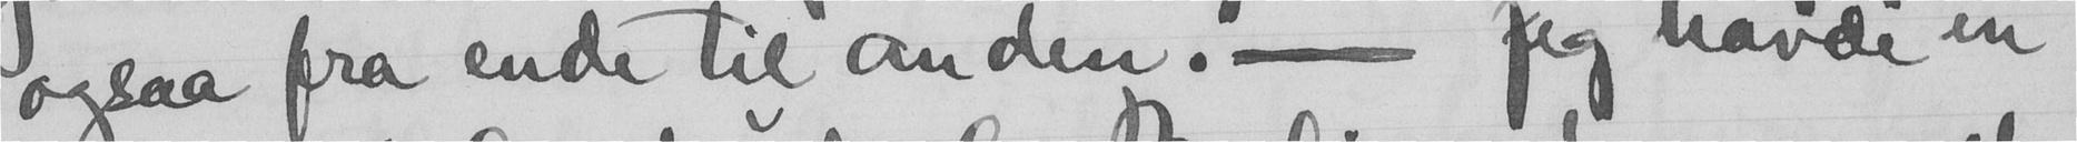ogsaa fra ende til anden. - Jeg havde en
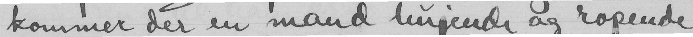kommer der en mand hujende og ropende
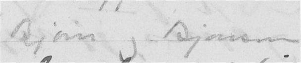Bjørn og Bjørnson
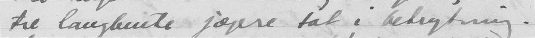til langbente jægere tat i betragtning.
